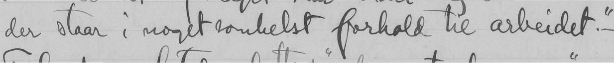der staar i noget somhelst forhold til arbeidet." -
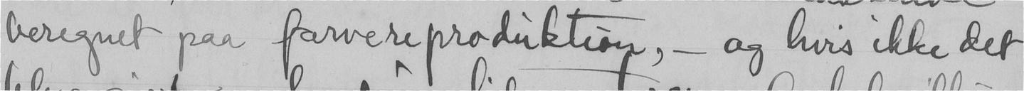beregnet paa farvereproduktion, - og hvis ikke det
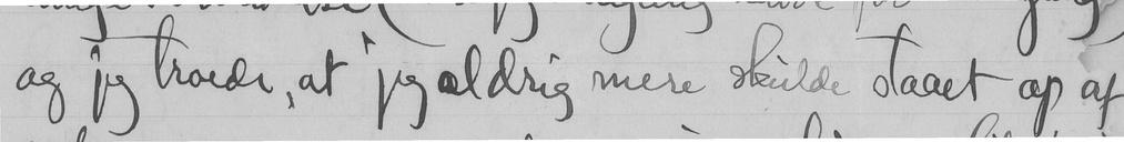og jeg troede, at jeg aldrig mere skulde staaet op af
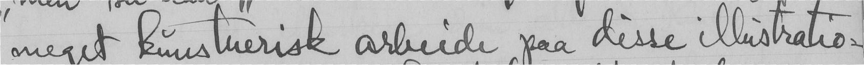meget kunstnerisk arbeide paa disse illustratio-
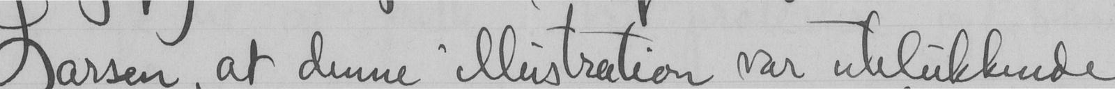Larsen, at denne illustration var utelukkende
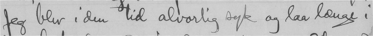Jeg blev i den tid alvorlig syk og laa længe i
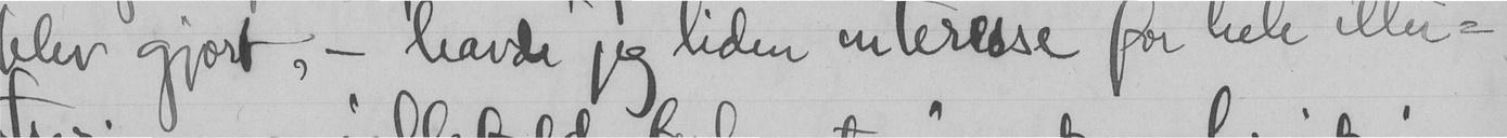blev gjort, - havde jeg liden interesse for hele illu-
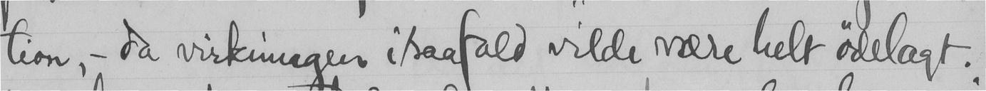tion - da virkningen isaafald vilde være helt ødelagt.
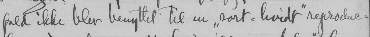fald ikke blev benyttet til en "sort-hvidt" reproduc-
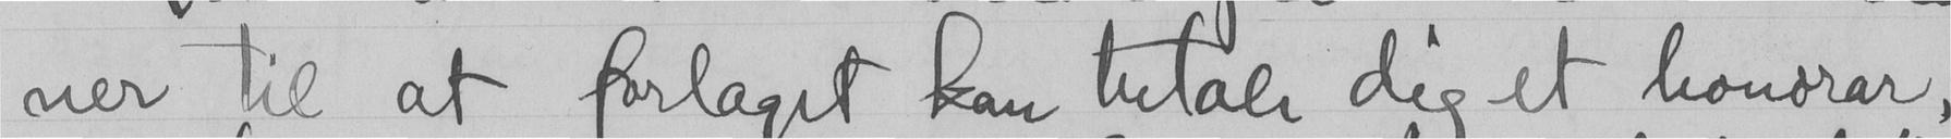ner til at forlaget kan betale dig et honorar,
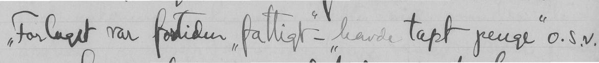"Forlaget var fortiden "fattigt" - havde tapt penge" o. s.v.
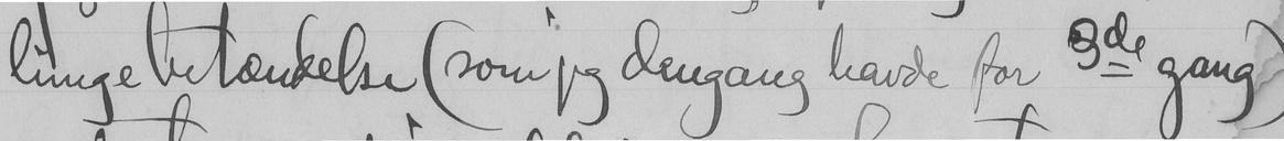lungebetændelse (som jeg dengang havde for 3de gang)
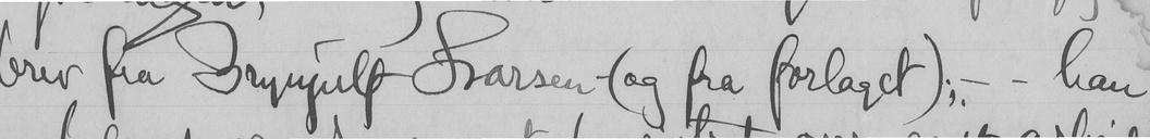brev fra Brynjulf Larsen (og far forlaget); - han
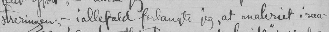streringen, - iallefald forlangte jeg, at maleriet i saa-
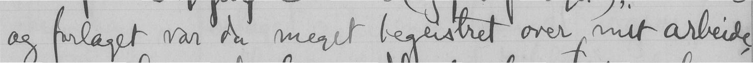og forlaget var da meget begeistret over mit arbeide
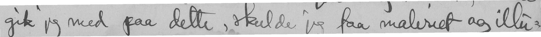gik jeg med paa dette, skulde jeg faa maleriet og illu-
